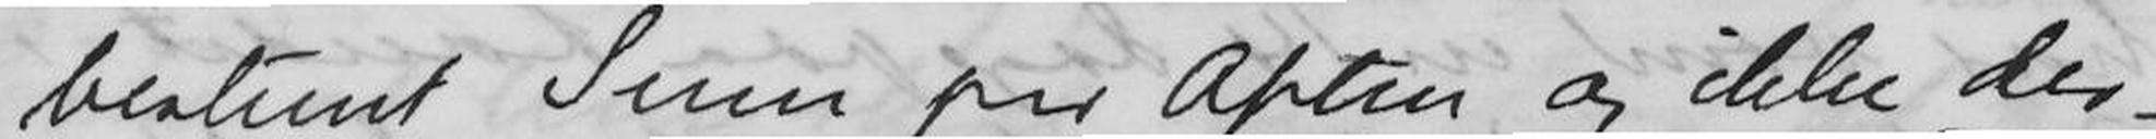bestemt Sum per Aften og ikke der-
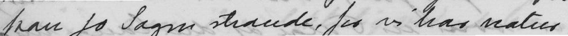kan jo Sagen strande, for vi har natur-
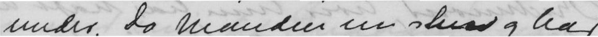under. Do Manden en stund og har
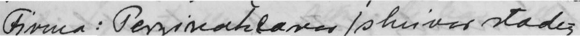Firma: Perzireaklaver) skriver stadig
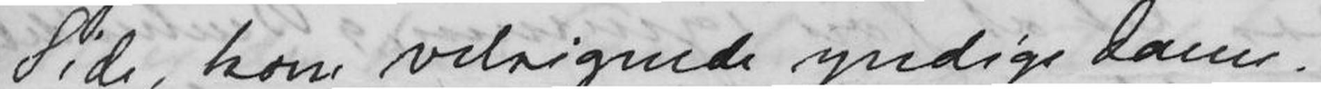Side, kom velsignede yndige Dame!
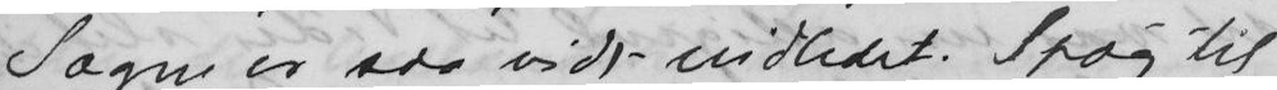Sagen er saa vidt indledet. Spøg til
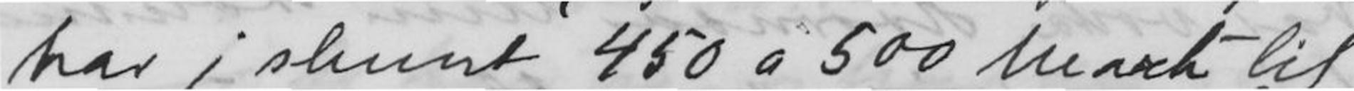har j skrevet 450 a 500 Marh til
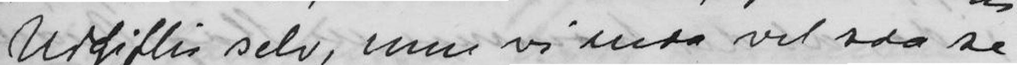Udgifter selv, men vi enda vil saa se
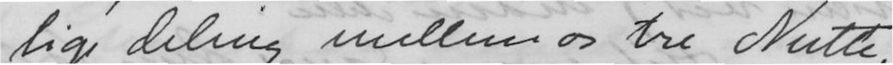lige deling mellem os tre Nutte-
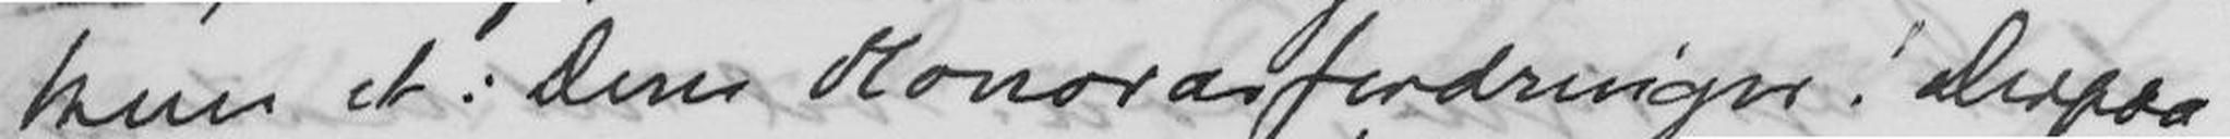kun et: Deres Honorarfordringer. Derpaa
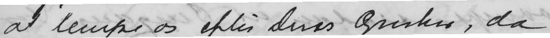at lempe os efter Deres Ønsker, da
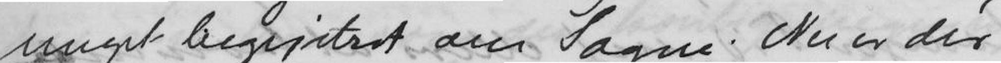meget begejstret om Sagen. Nu er der
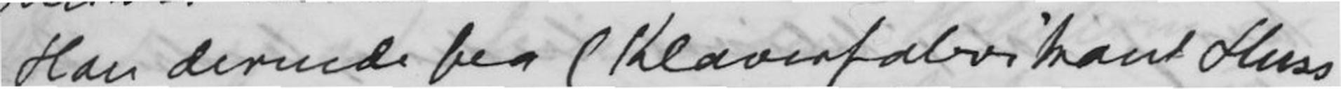Han dernede paa (Klaverfabrikans Huss
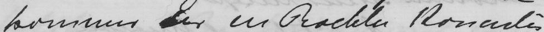kommer der en Raekke Koncerter
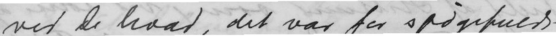-ved De hvad, det var for spøgefuldt
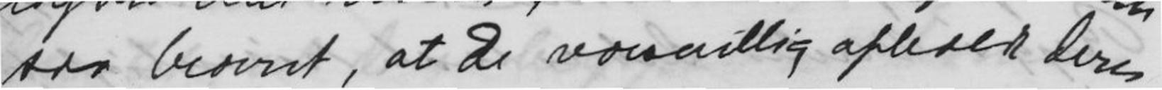saa beaeret, at De vaesentlig afholdt Deres
